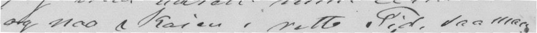og naa Kaien i rette Tid, saa maa
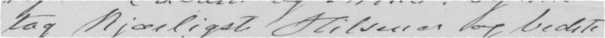tag kjærligst Hilsener og bedste
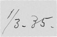1/3. 35.
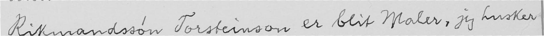Rikmandssøn Torsteinson er blit Maler, jeg husker
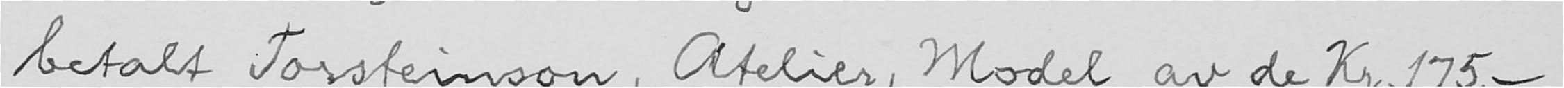betalt Torsteinson, Atelier, Model av de Kr. 175. -
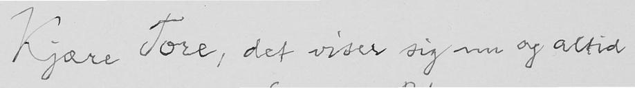Kjære Tore, det viser sig nu og altid
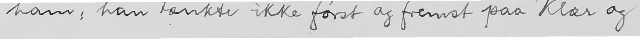ham, han tænkte ikke først og fremst paa Klær og
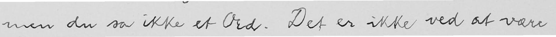men du sa ikke et Ord. Det er ikke ved at være
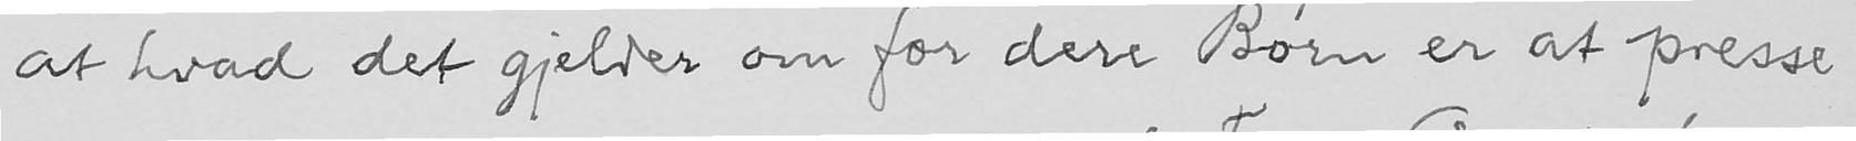at hvad det gjelder om for dere Børn er at presse
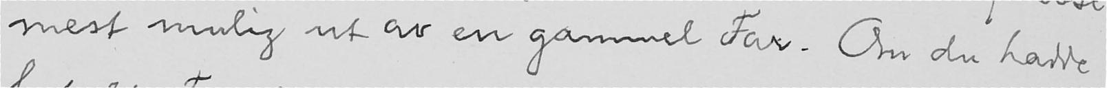mest mulig ut av en gammel Far. Om du hadde
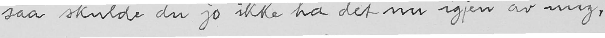saa skulde du jo ikke ha det nu igjen av mig,
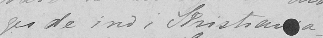ges de ind i Kristiania-
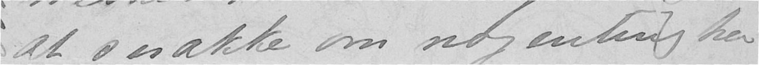at snakke om nogenting her
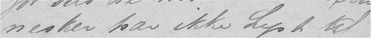nesker har ikke Lyst til
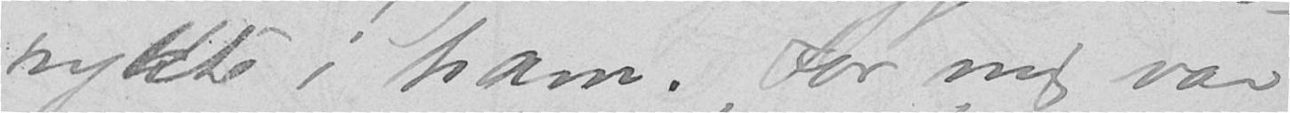rykte i ham. For mig var
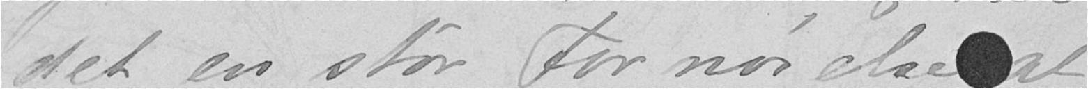det en stor Fornøielse at
